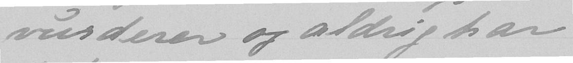vurderer og aldrig har
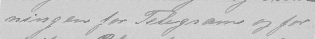ningen for Telegram og for
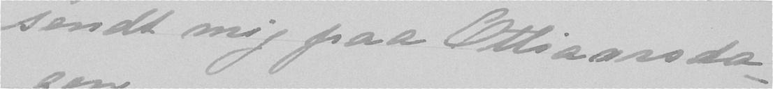sendt mig paa Ottiaarsda-
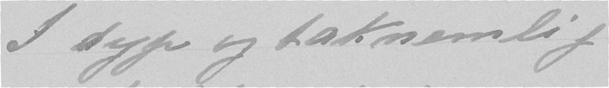I dyp og taknemlig
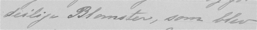deilige Blomster, som blev
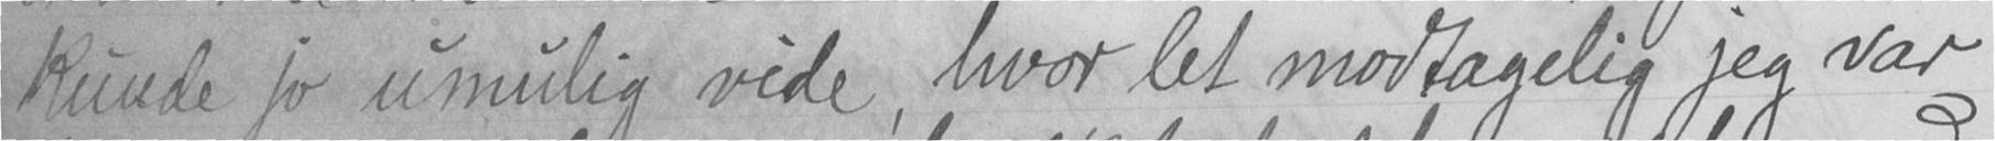kunde jo umulig vide, hvor let modtagelig jeg var
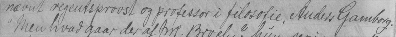nævnt regentsprovst og profissor i filosofie, Anders Gamborg.
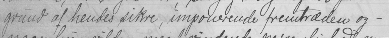grund af hendes sikre, imponerende fremtræden og -
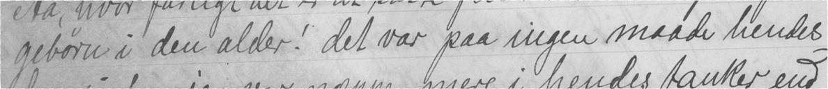gebørn i den alder! det var paa ingen maade hendes
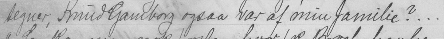tegner, Knud Gamborg ogsaa var af min familie?...
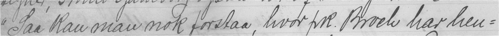"Saa kan man nok forstaa, hvor frk. Broch har hen-
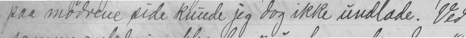paa mødrene side kunde jeg dog ikke undlade. Ved
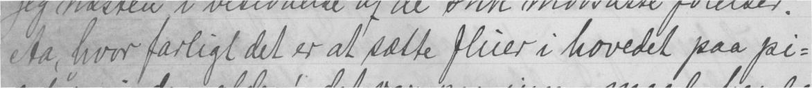Aa, hvor farligt det er at sætte fluer i hovedet paa pi-
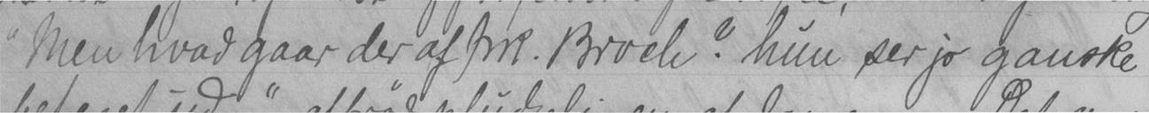"Men hvad gaar der af frk. Broch? hun ser jo ganske
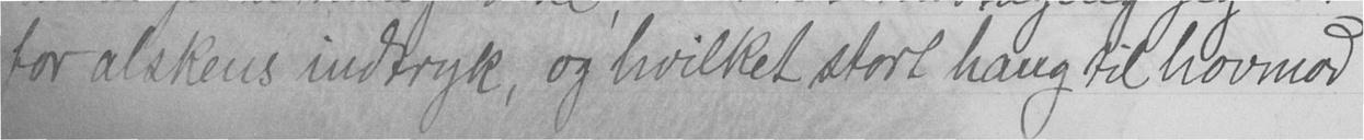for alskens indtryk, og hvilket stort hang til hovmod
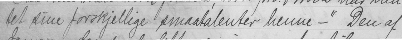tet sine forskjellige smaatalenter henne -" Den af
